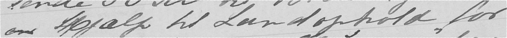en Hjælp til Landophold for
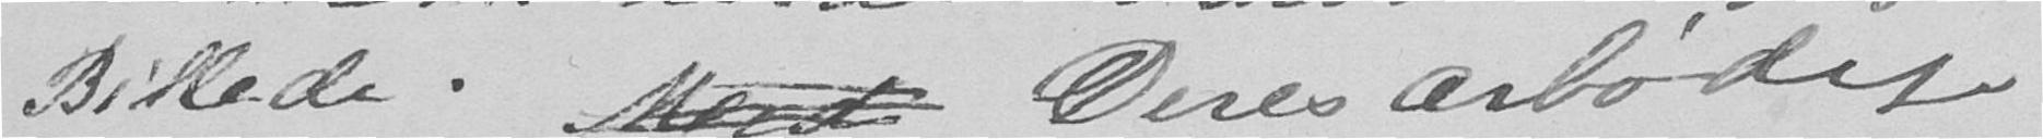Billede. Med Deres arbødige
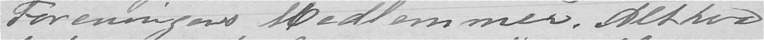Foreningens Medlemmer. Alt hvad
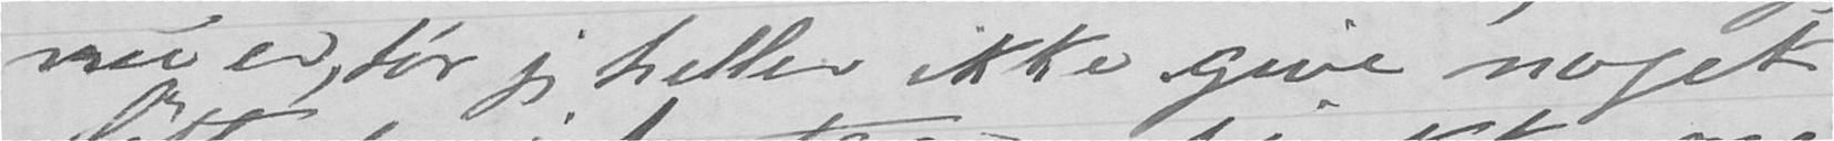nu er, tør jeg heller ikke give noget
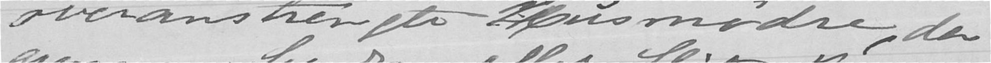overanstrengte Husmødre, der
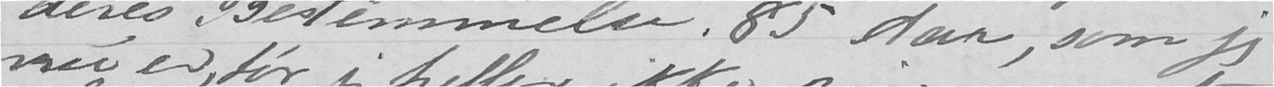deres Bestemmelse. 85 Aar, som jeg
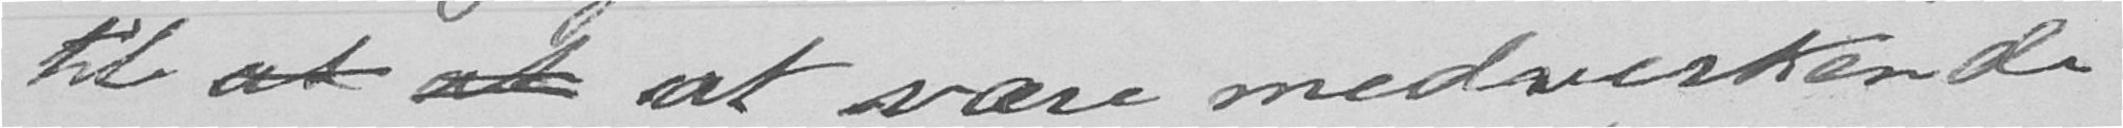til at at at være medverkende
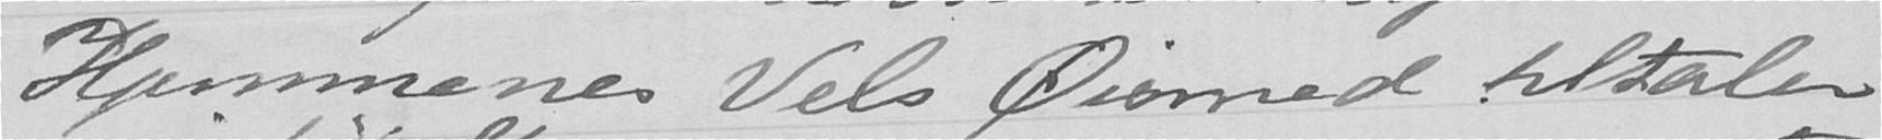Hjemmenes Vels Øiemed tiltaler
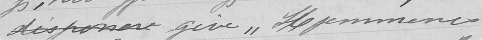desponere give "Hjemmenes
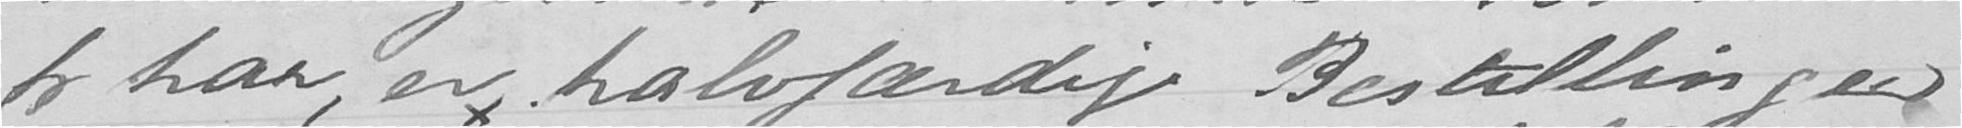jeg har er, halvfærdige Bestillinger
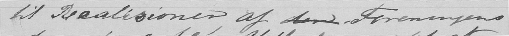til Realisioner af den Foreningens
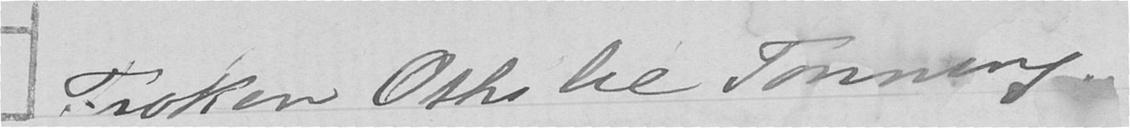Frøken Othilie Tonning.
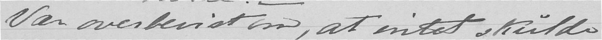Vær overbevist om, at intet skulde
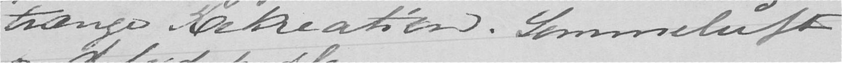trænge Rekreatio.. Sommeluft
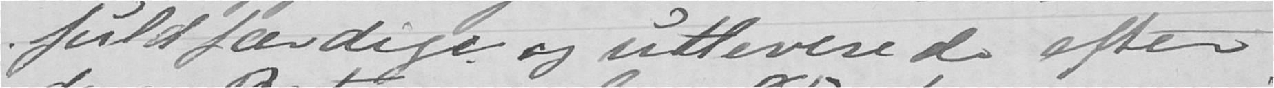fuldferdige og utleverede efter
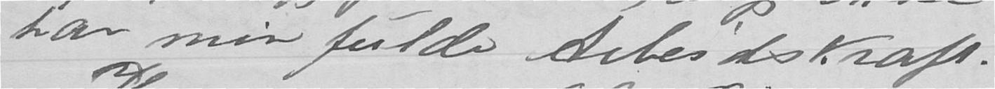har min fulde Arbeidskraft.
